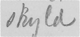skyld
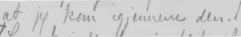at jeg kom igjennem den.
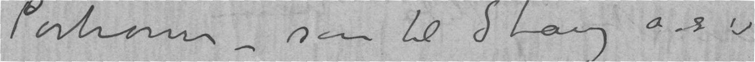Portioner – Som til Stang o.s.v
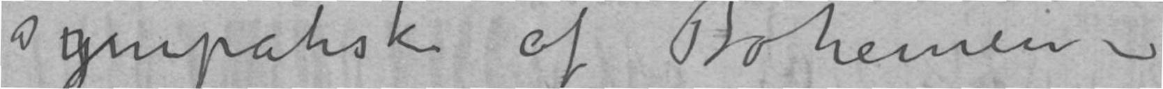sympatiske af Bohemer–
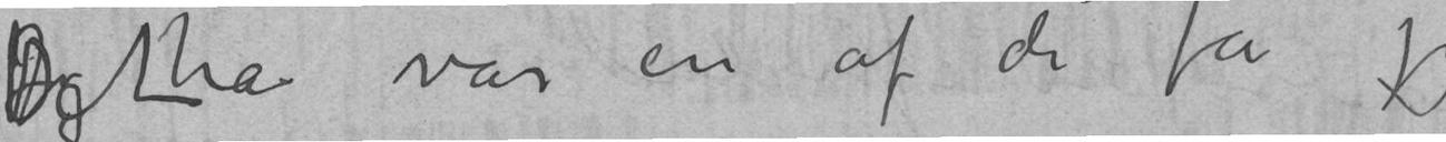Jeg haDet var en af de få jeg
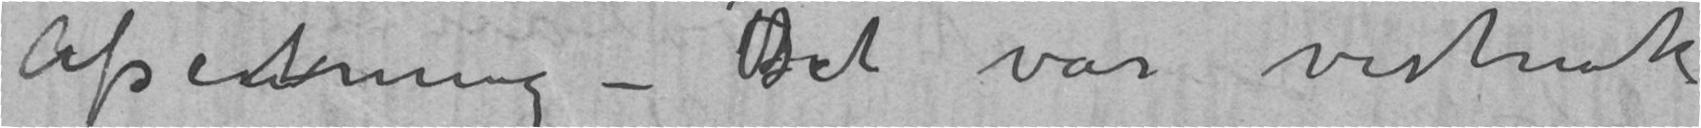Afsetning – Det var vistnok
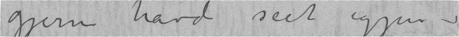gjerne havde seet igjen –
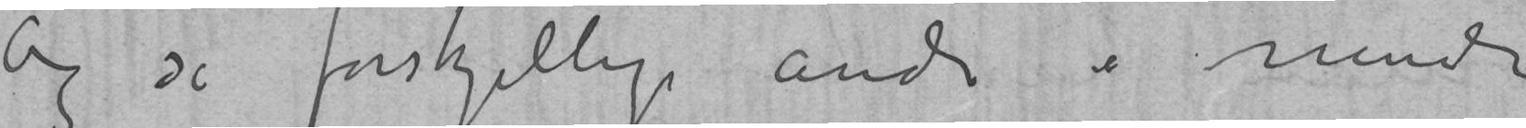og så forskjellige andre i mindre
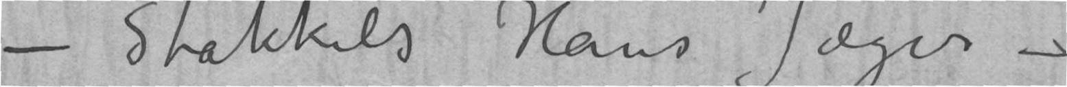– Stakkels Hans Jæger –
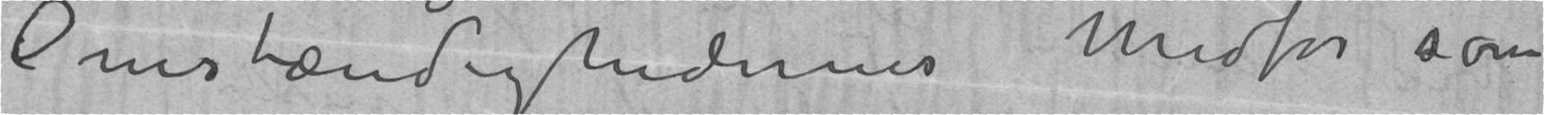Omstændighedernes Medfør som
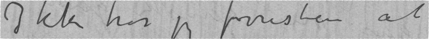Ikke tror jeg forresten at
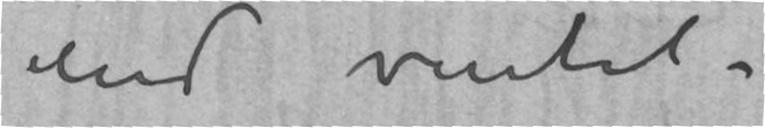end ventet –
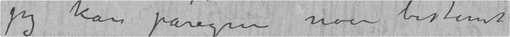jeg kan påregne noen bestemt
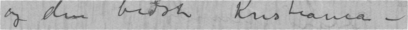og den bedste Kristiania-
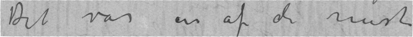Det var en af de mest
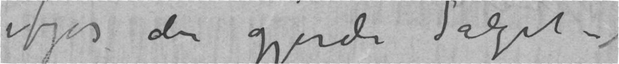ifjor d gjorde Salget
